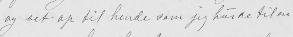og set op til hende som jeg burde til en
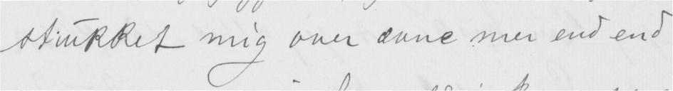strukket mig over ævne mer end end
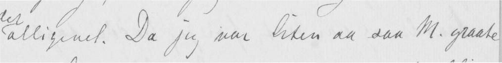alligevel. Da jeg var liten aa saa M. graate
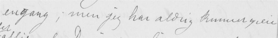engang; men jeg har aldrig kunnet greie
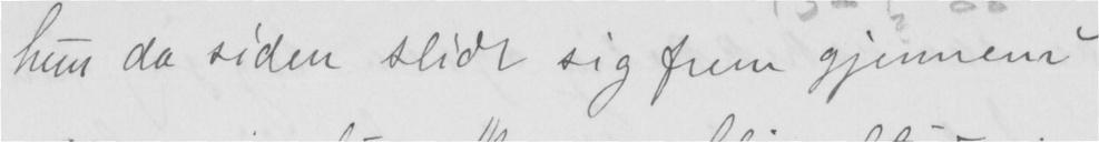hun da siden slidt sig frem gjennem
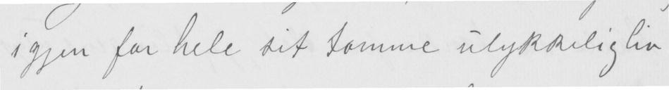igjen for hele sit tomme ulykkelig liv
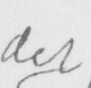det
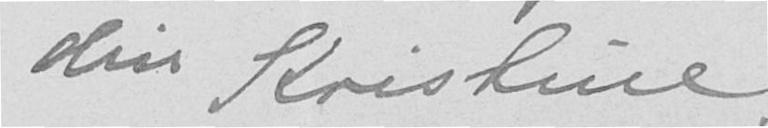din Kristine
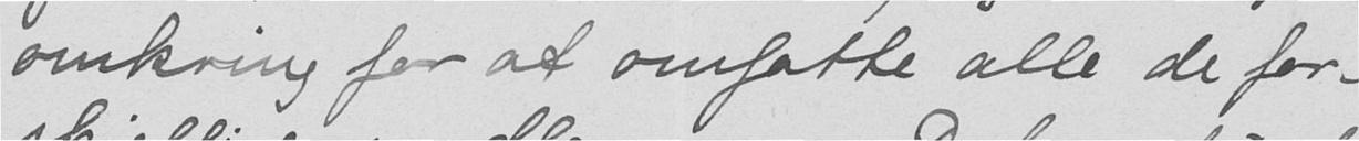omkring for at omfatte alle de for-
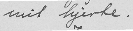mit hjerte.
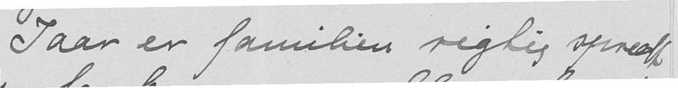I aar er familien rigtig spredt
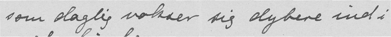som daglig vokser sig dybere ind i
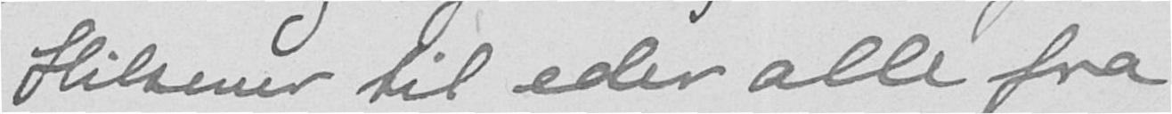Hilsener til eder alle fra
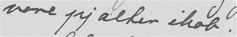vore pjalter ihob.
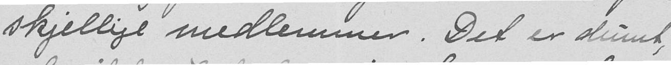skjellige medlemmer. Det er dumt,
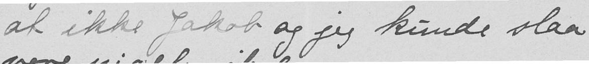at ikke Jakob og jeg kunde slaa
